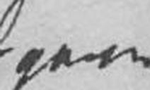gaves
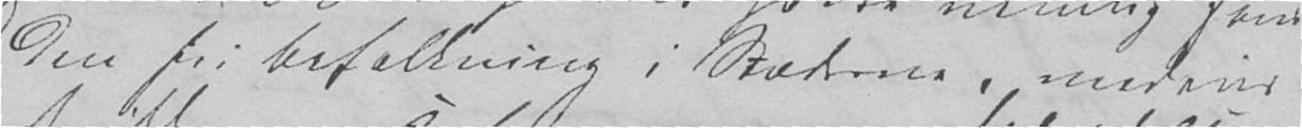den fri Befolkning i Staterne, medens
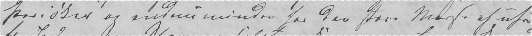Perioker og endnu mindre for den store Masse af ufrie
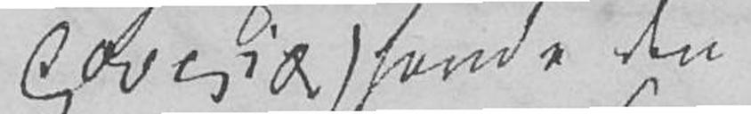X) havde den
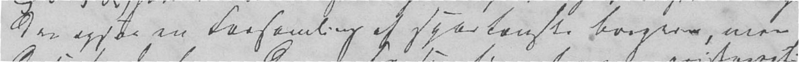der ogsaa en Forsamling af spartanske Borgere, men
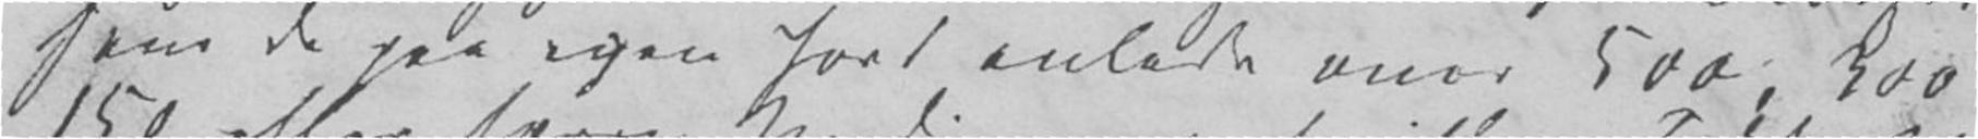som de paa egen Jord avlede over 500, 300
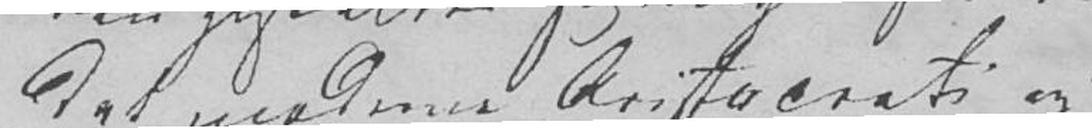det moderne Aristocrati og
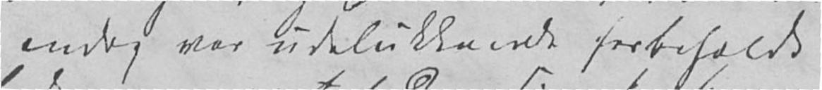endog var udelukkende forbeholdt
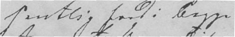sentlig fordi begge
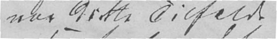var dette Tilfælde
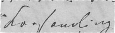Forsamling
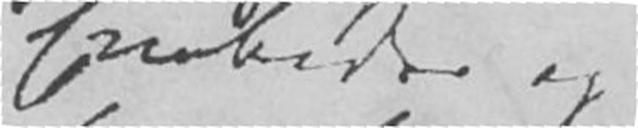Embeder og
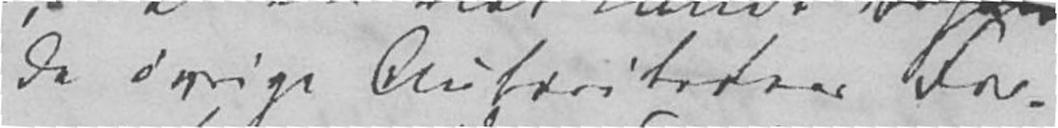de øvrige Autoriteters For-
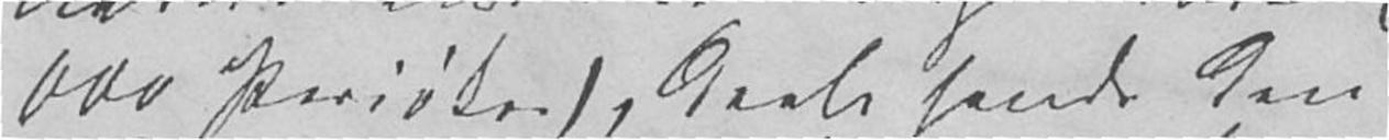000 Perioker), deels havde den
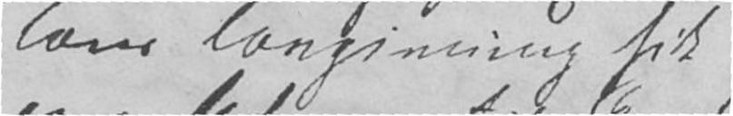lons Lovgivning fik
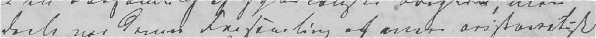deels var denne Forsamling af mere aristocratisk
